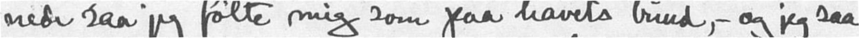nede saa jeg følte mig som paa havets bund,- og jeg saa
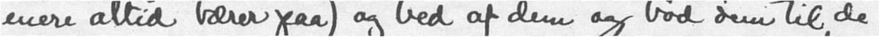enere altid bærer paa) og bed af dem og bød dem til de
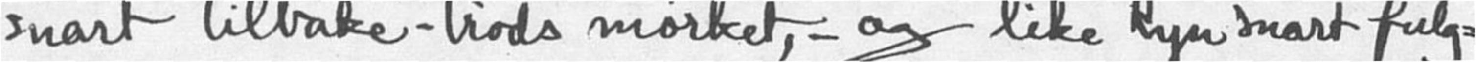snart tilbake - trods mørket, - og like lynsnart fulg-
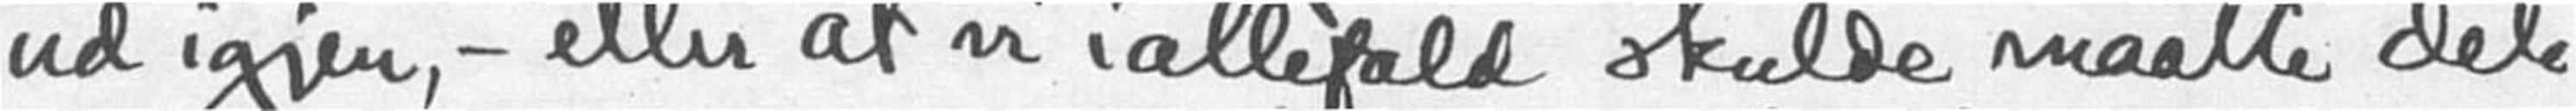ud igjen, - eller at vi iallefald skulde maate dele
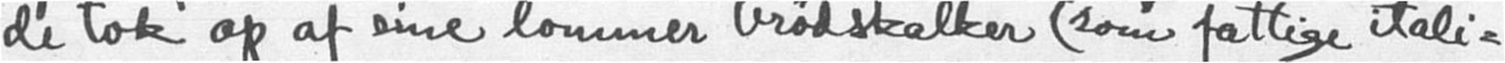de tok op af sine lommer brødskalker (som fattige itali-
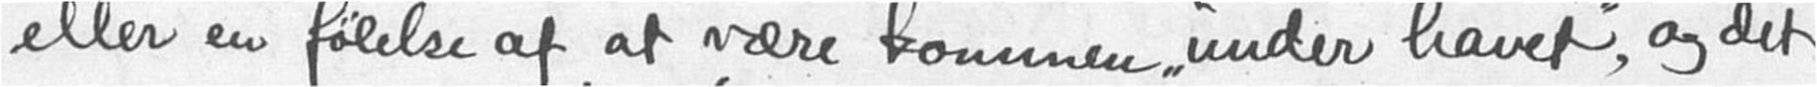eller en følelse af at være kommen "under havet" og det
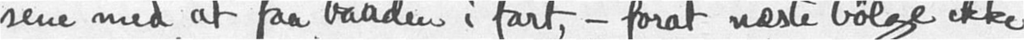sene med at faa baaden i fart, - forat næste bølge ikke
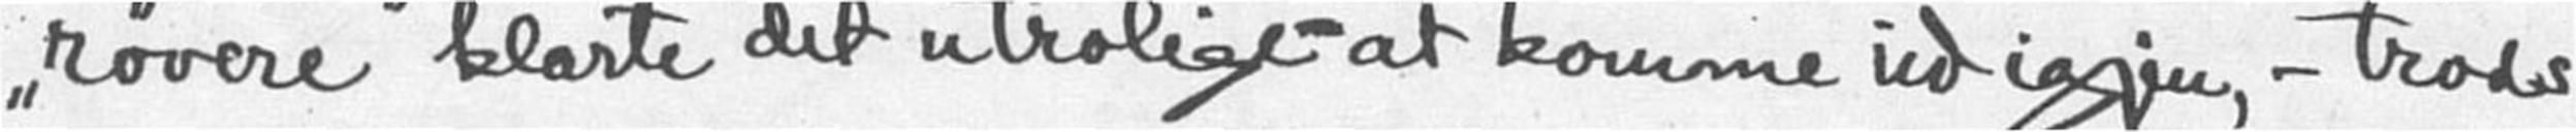"røvere" klarte det utrolige - at komme ud igjen, - trods
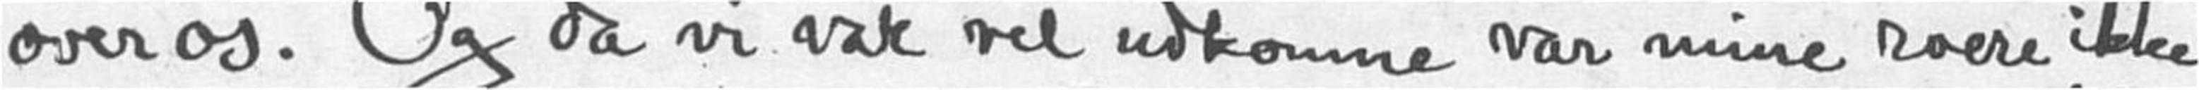over os. Og da vi var vel udkomne var mine roere ikke
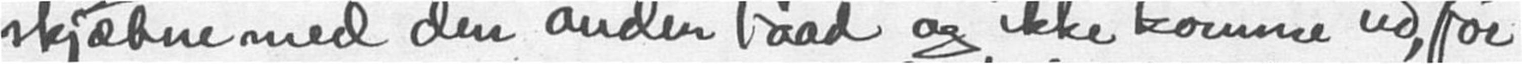skjæbne med den anden baad og ikke komme ud, for
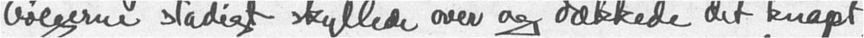bølgerne stadigt skyllede over og dækkede det knapt
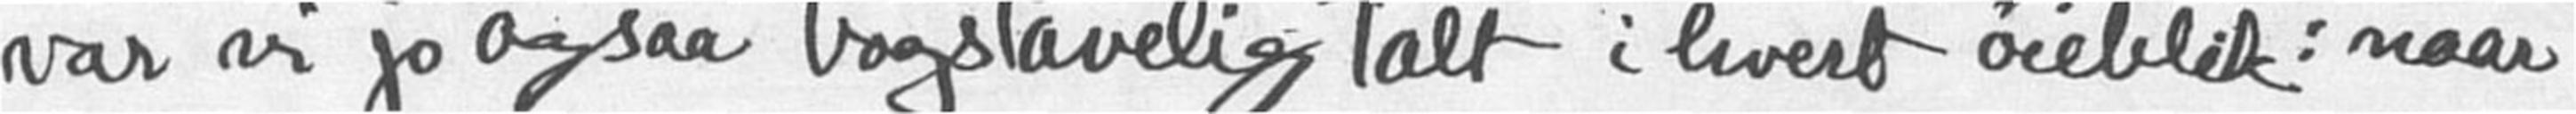var vi jo ogsaa bogstavelig talt i hvert øieblik: naar
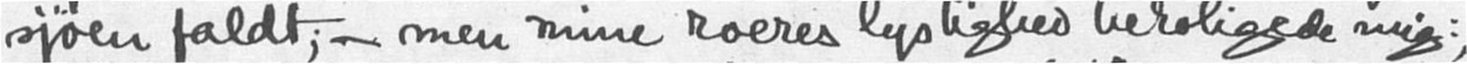sjøen faldt; - men mine roeres lystighed beroligede mig: -
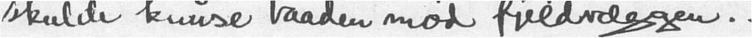skulde knuse baaden mod fjeldvæggen. -
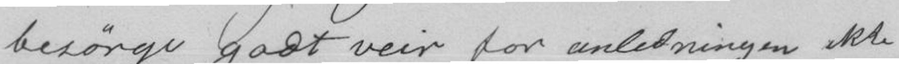besørge godt veir for anldningen ikke
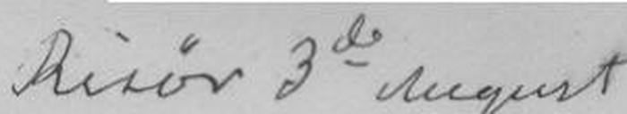Risør 3de August
